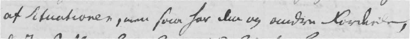af situationen, men saa har den og andre Fordele,
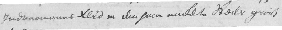Indvaanernes Flid er den paa enkelte Stæder giort
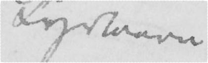Lystaarn
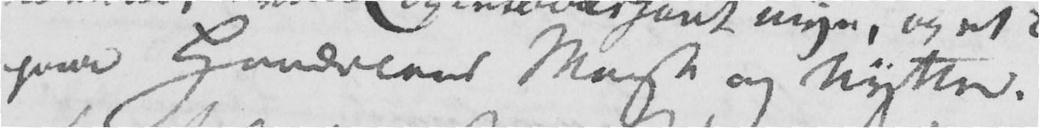paa Handelens Magt og Nytte.
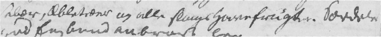sebær, Æbletræer og alle slags Havefrugter. Særdeles
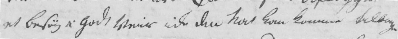et besøg i godt Veir icke den Nat kan komme tilbage
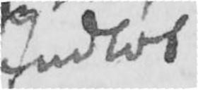indløb
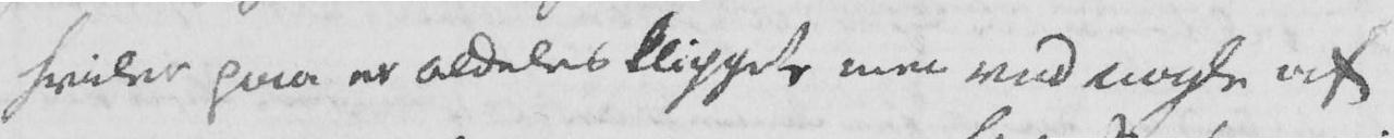hviler paa er aldeles klippete men ved nogle af
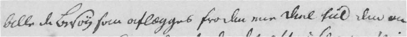Alle de Besøg som aflægges fra den ene Deel til den an-
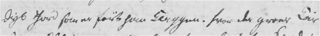dyb Jord som er ført paa Klippen. Hvor der groer Kir-
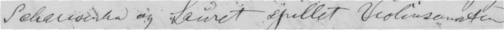Scharwenka og Lauret spillet Violinsonaten
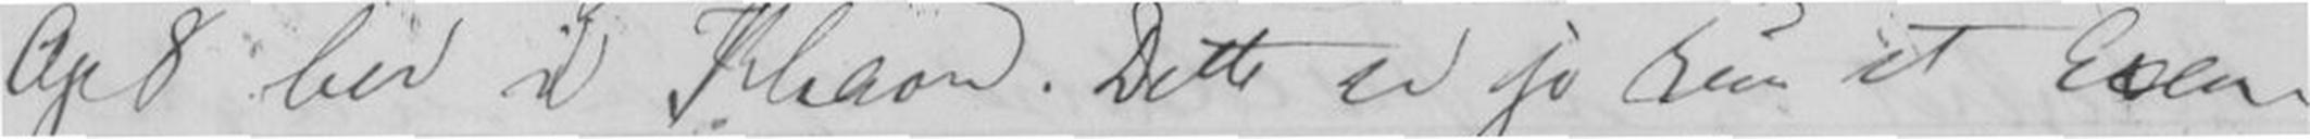Op 8 her i Khavn. Dette er jo kun et Exem-
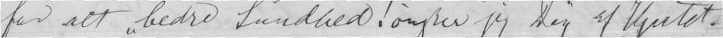for alt bedre Sundhed! ønsker jeg Dig af Hjertet.
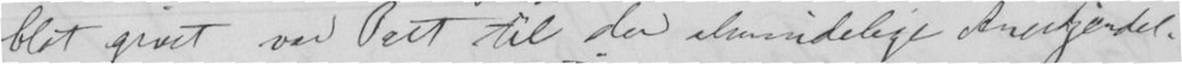blot givet vor Part til den almindelige Anerkjendel-
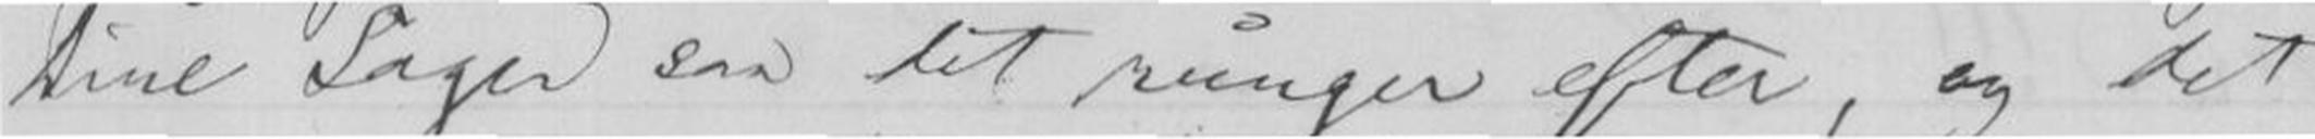Dine Sager saa det runger efter, og det
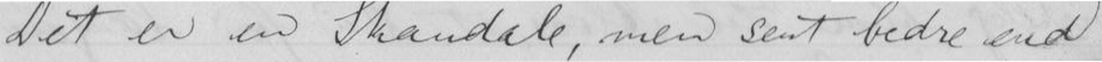Det er en Skandale, men sent bedre end
